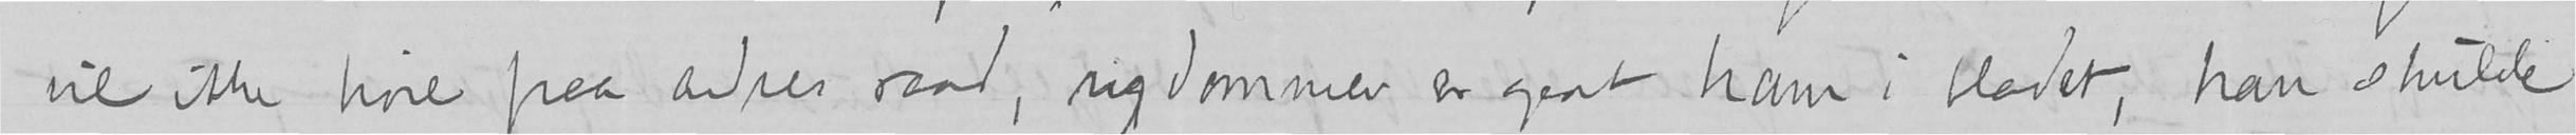vil ikke høre paa andres raad, rigdommen er gaat ham i blodet, han skulde
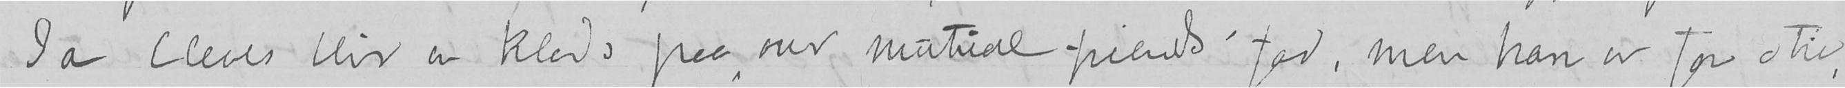Ja Cleves blir en klods paa "our mutual friends" fod, men han er for stiv,
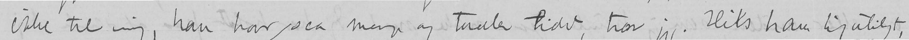ikke til mig, han har saa mange og taaler lidet, tror jeg. Hils ham hjerteligt,
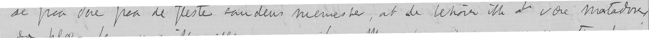se paa dere paa de fleste aandens mennesker, at de behøver ikke at være matadorer,
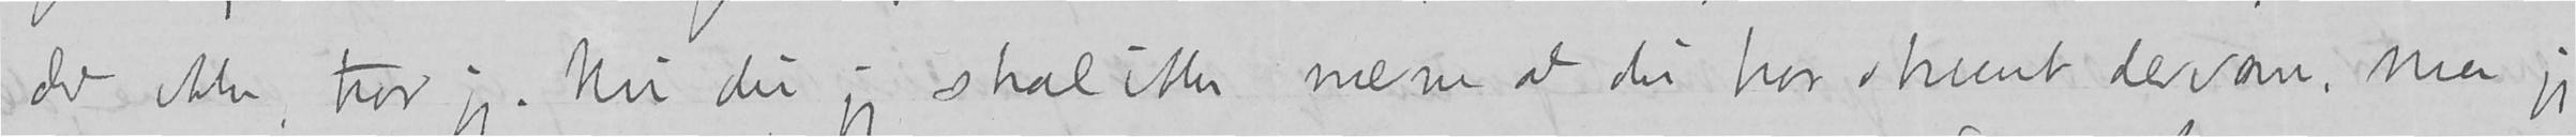det ikke, tror jeg. Nu du jeg skal ikke nævne at du har skrevet derom, men jeg
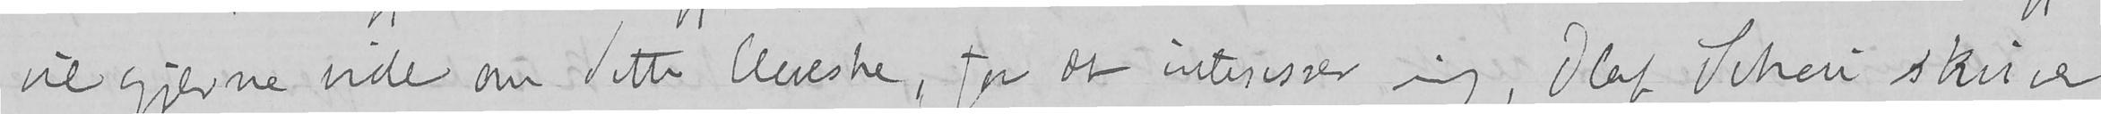vil gjerne vide om dette Cleveske, for det interesser mig, Olaf Schou skriver
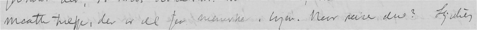maatte træffe, der er vel faa mennesker i byen. Naar reiser dere? Hjertelig
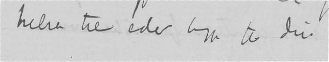hilsen til eder begge fra din
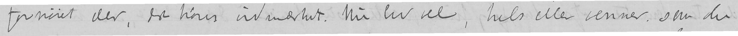fornøiet der, det høres udmærket. Nu lev vel, hils alle venner som du
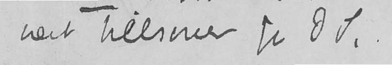vært hilsener fra J S.
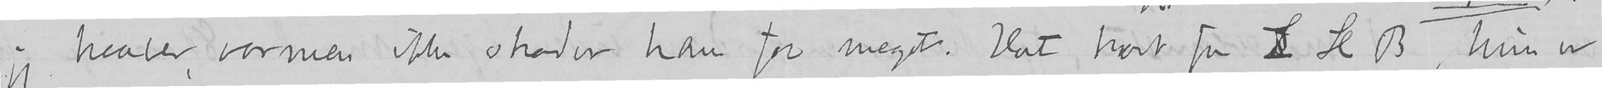jeg haaber, varmen ikke skader ham for meget. Hat hørt fra L H B, hun er
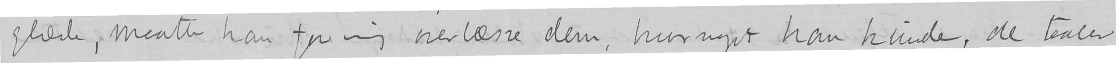glæde, maatte han for mig overlæsse dem, hvormeget han kunde, de taaler
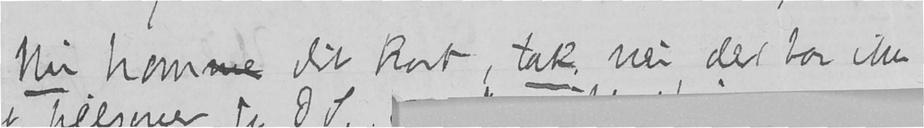Nu komme dit kort, tak nei der bor ikke
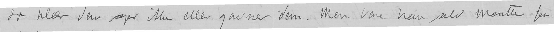det klær dem sogar ikke eller gavner dem. Men bare han selv maatte faa
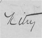Kitty
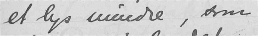et lys mindre, som
